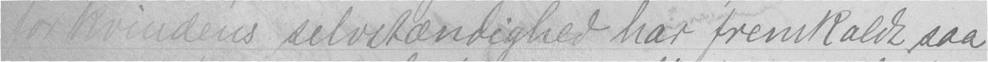for kvindens selvstændighed har fremkaldt saa
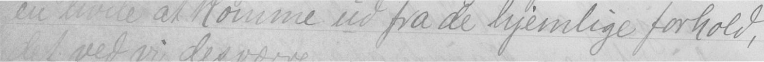en hvile at komme ud fra de hjemlige forhold,
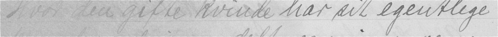Hvor den gifte kvinde har sit egentlige
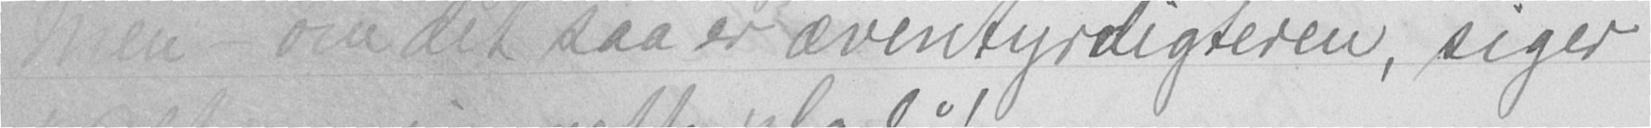Men - om det saa er æventyrdigteren, siger
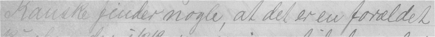Kanske finder nogle, at det er en forældet
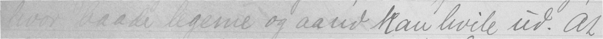hvor baade legeme og aand kan hvile ud. At
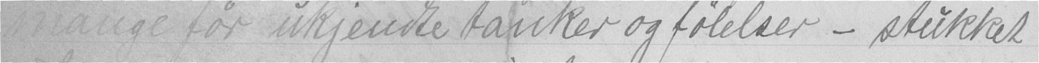mange før ukjendte tanker og følelser - stukket
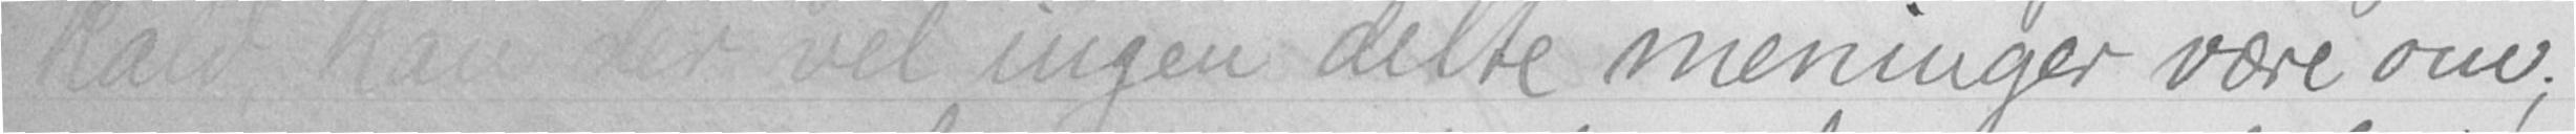kald, kan der vel ingen delte meninger være om;
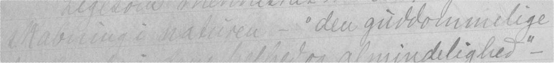skabning i naturen - "den guddommelige
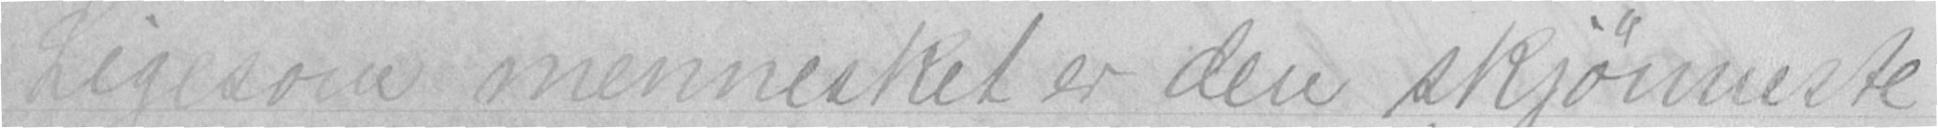Ligesom mennesket er den skjønneste
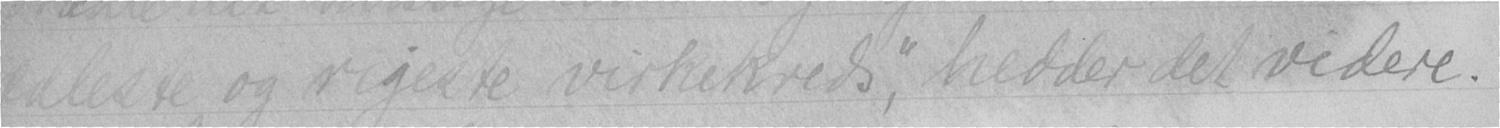ædleste og rigeste virkekreds," hedder det videre.
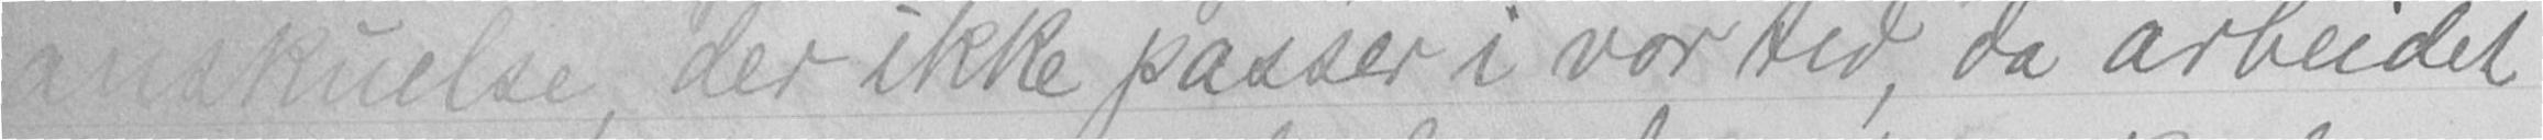anskuelse, der ikke passer i vor tid, da arbeidet
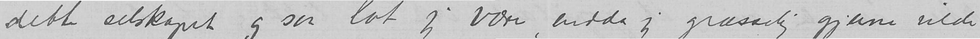dette selskapet og saa lot jeg være, endda jeg græselig gjerne vilde
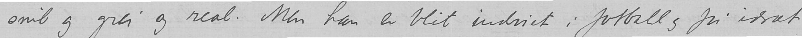snil og grei og real. Men han er blit indviet i fotball og fri idræt
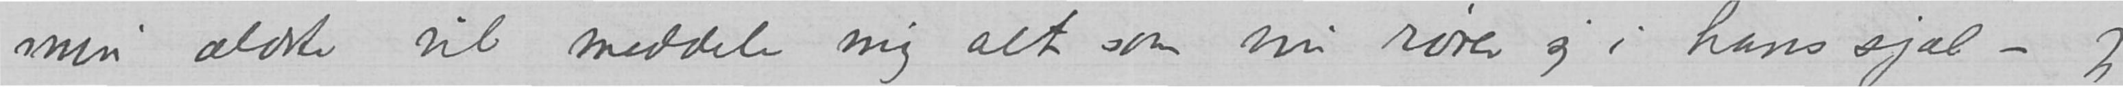min ældste vil meddele mig alt som nu rører sig i hans sjæl – jeg
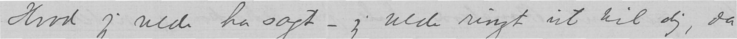Hvad jeg vilde ha sagt – jeg <vilde> ringt ut til dig, da
